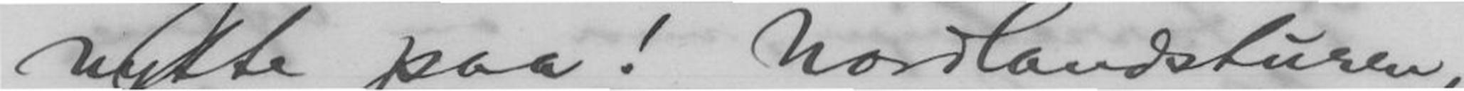nytte paa! Nordlandsturen,
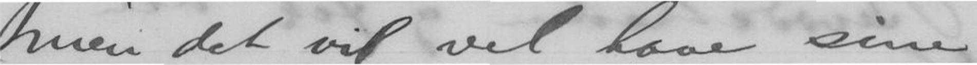men det vil vel have sine
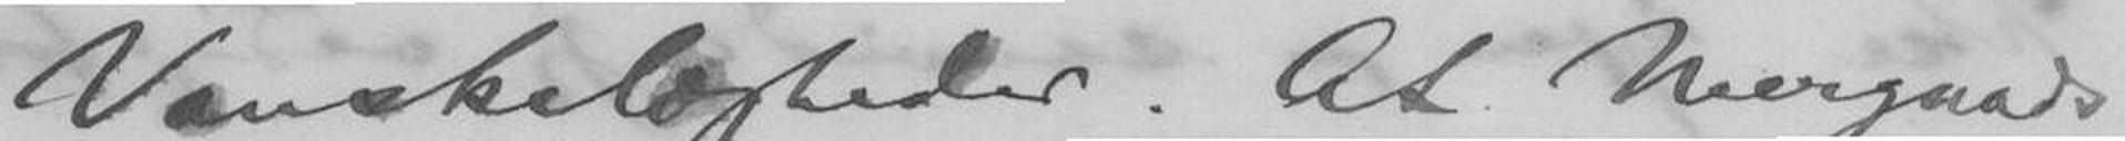Vanskeligheder. At Neergaards
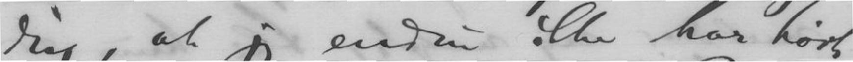dig, at jeg endnu ikke har hørt
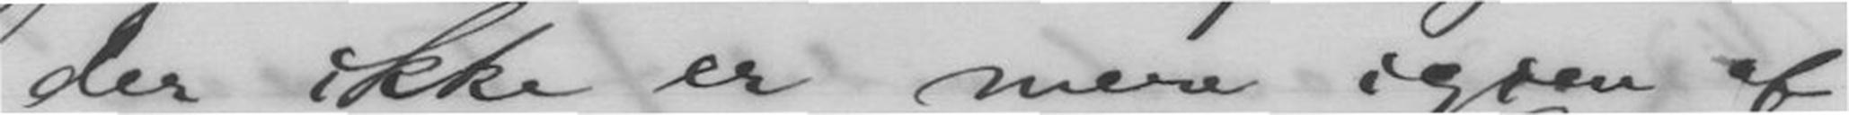der ikke er mere igjen af
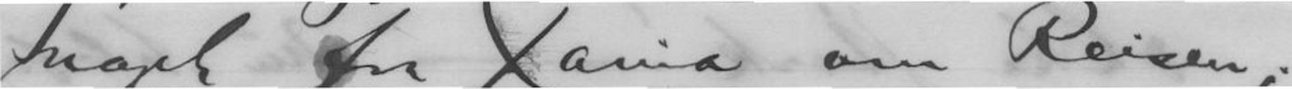noget fra Xania om Reisen;
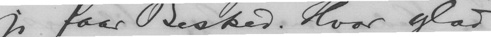jeg faar Besked. Hvor glad
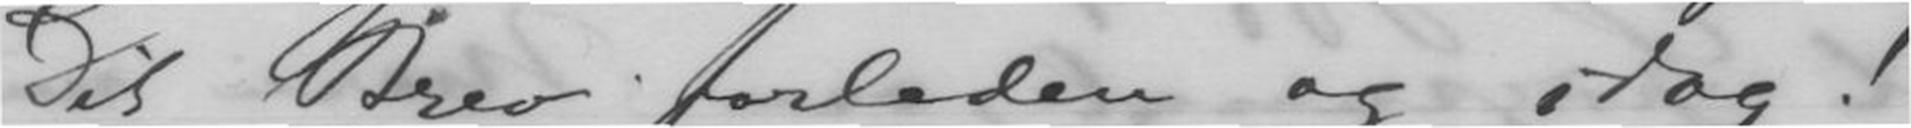Dit Brev forleden og idag!
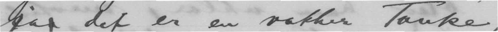ja det er en vakker Tanke,
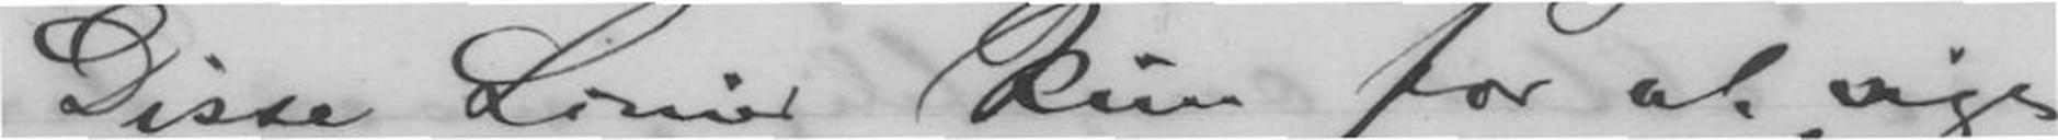Disse Linier kun for at sige
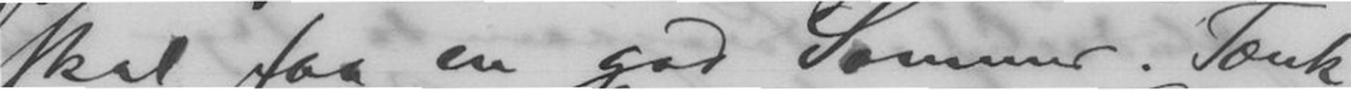skal faa en god Sommer. Tænk,
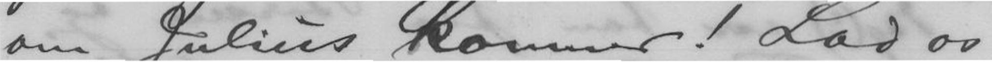om Julius kommer! Lad os
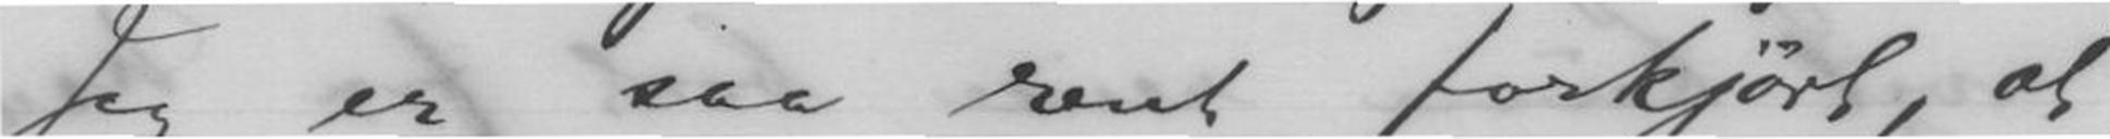Jeg er saa rent forkjørt, at
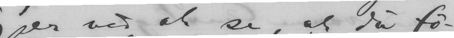jeg er ved at se, at du fø-
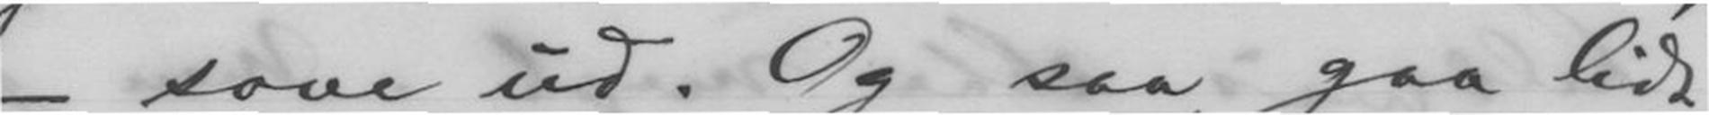- sove ud. Og saa gaa lidt.
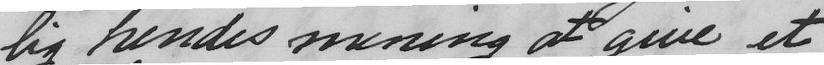lig hendes mening at give et
